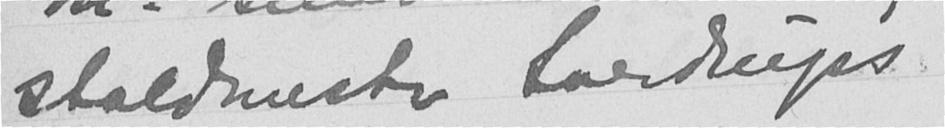staldmester Sverdrups
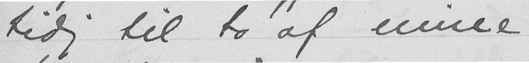tidig til to af mine
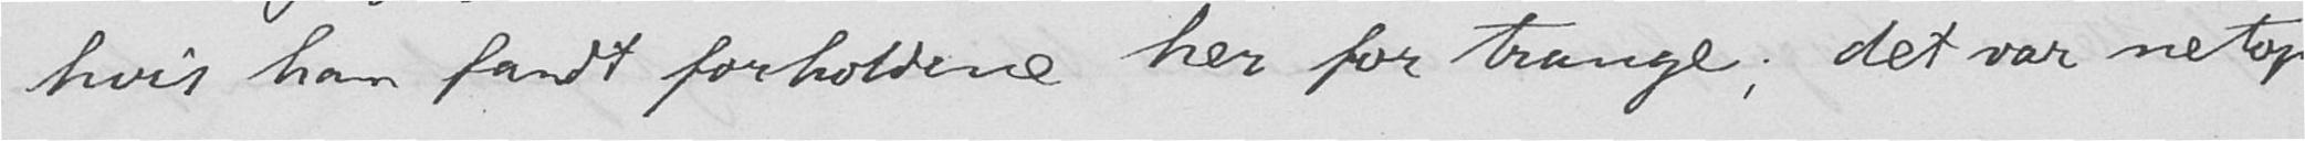hvis han fandt forholdene her for trange; det var netop
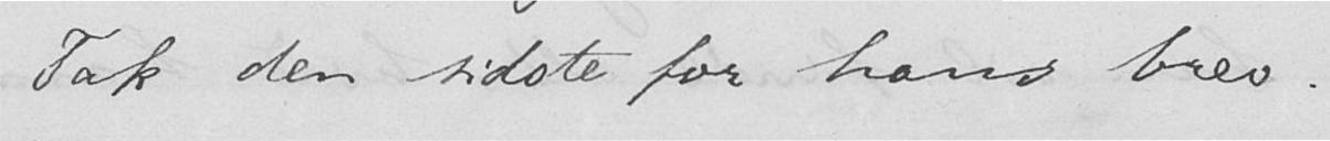Tak den sidste for hans brev.
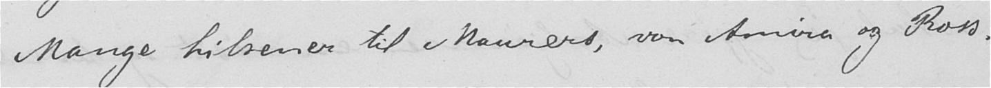Mange hilsener til Maurers, von Amira og Ross.
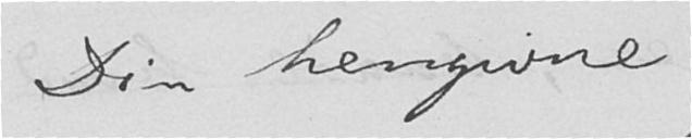Din hengivne
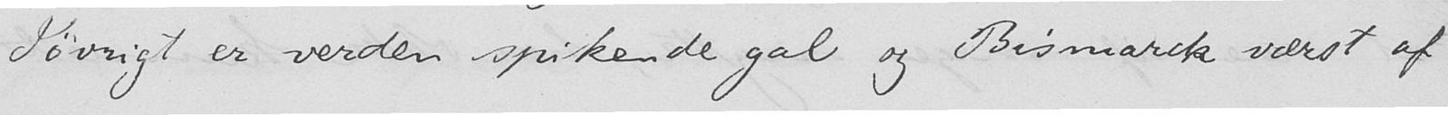Iøvrigt er verden spikende gal og Bismarck værst af
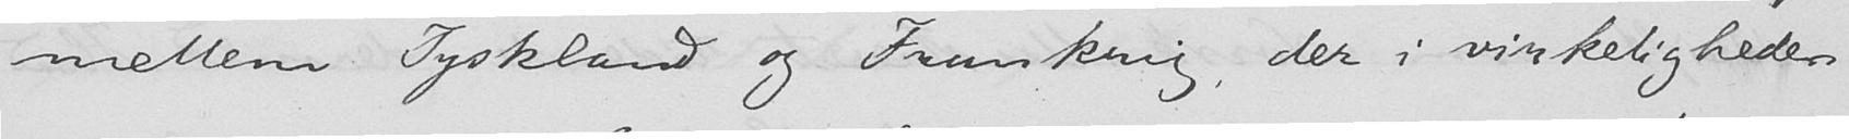mellem Tyskland og Frankrig, der i virkeligheden
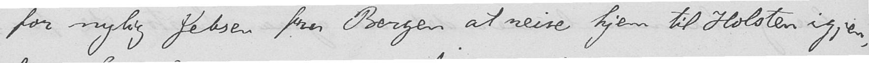for nylig Jebsen fra Bergen at reise hjem til Holsten igjen,
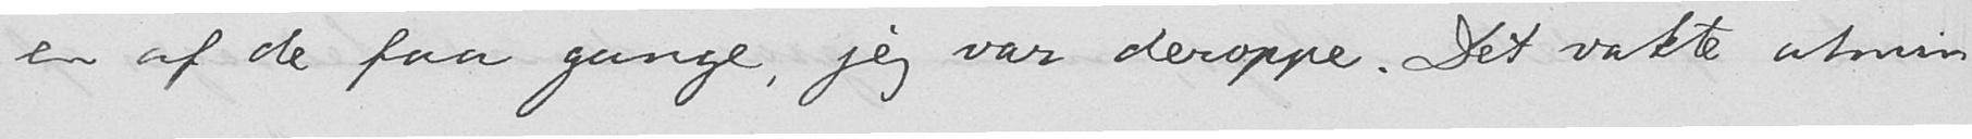en af de faa gange, jeg var deroppe. Det vakte almin-
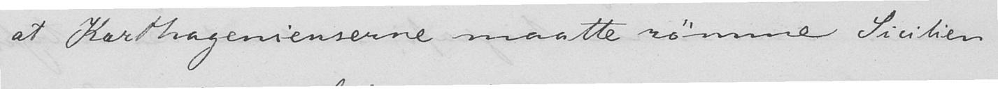at Karthagenienserne maatte rømme Sicilien.
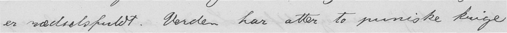er rædselsfuldt. Verden har atter to puniske krige
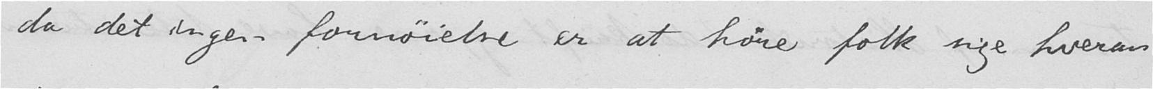da det ingen fornøielse er at høre folk sige hveran-
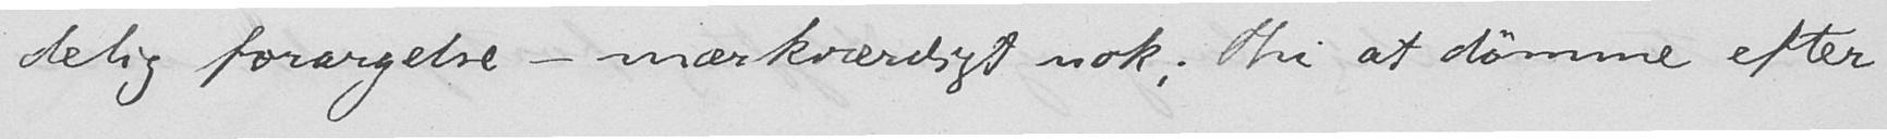delig forargelse - mærkværdigt nok; thi at dømme efter
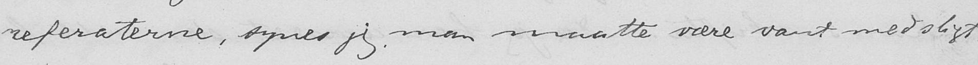referaterne, synes jeg man maatte være vant med sligt.
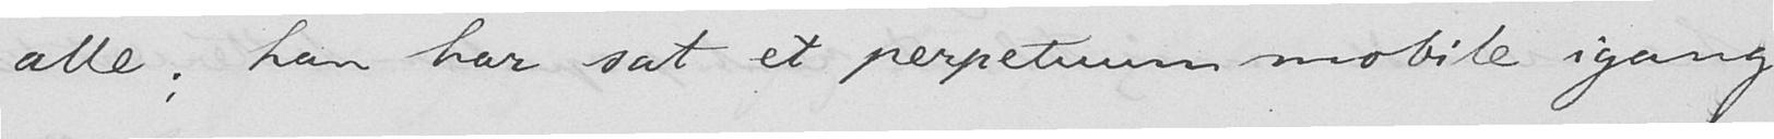alle; han har sat et perpetuum mobile igang
